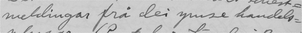meldingar frå dei ymse handels-
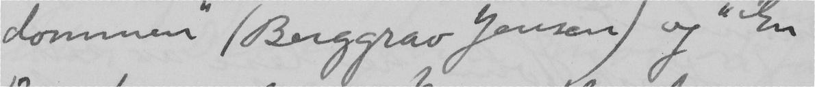dommen" (Berggrav Jensen) og "En
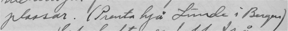plassar. (Prenta hjå Lunde i Bergen).
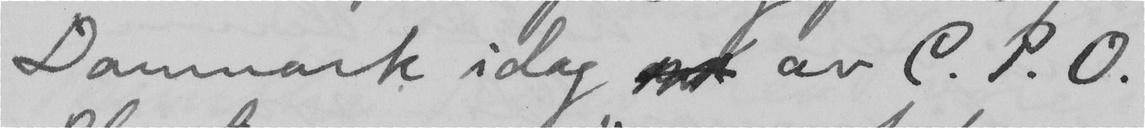Danmark idag  av C.P.O.
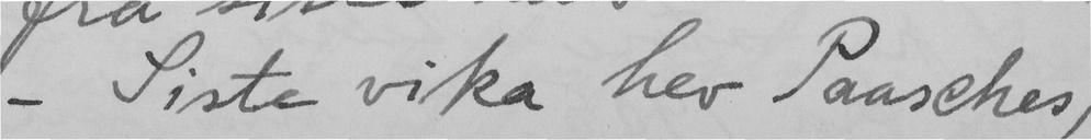– Siste vika hev Paasches,
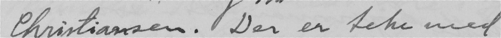Christiansen. Der er teke med
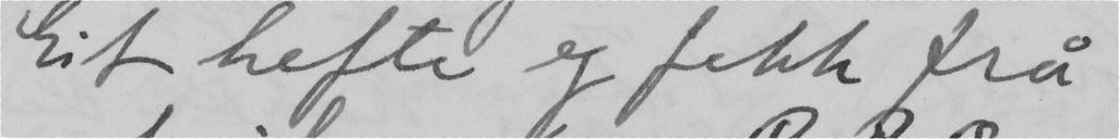Eit hefte eg fikk frå
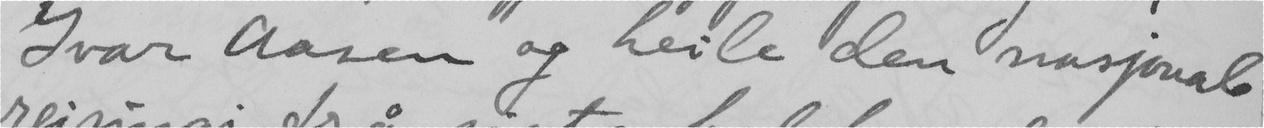Ivar Aasen og heile den nasjonale
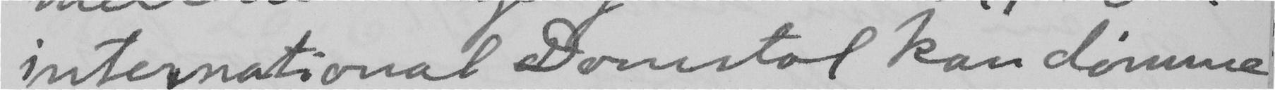international Domstol kan dömme
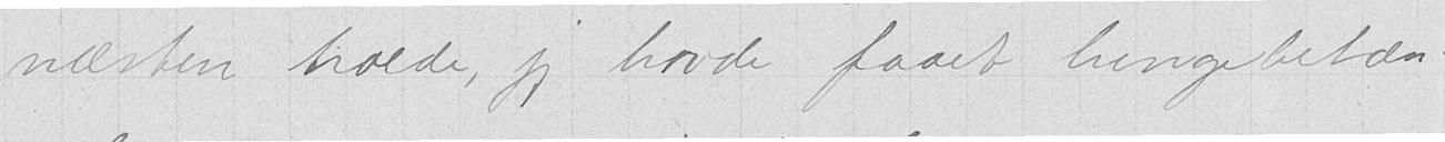næsten troede, jeg havde faaet lungebetæn-
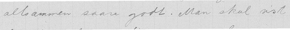altsammen saare godt. Man skal vist
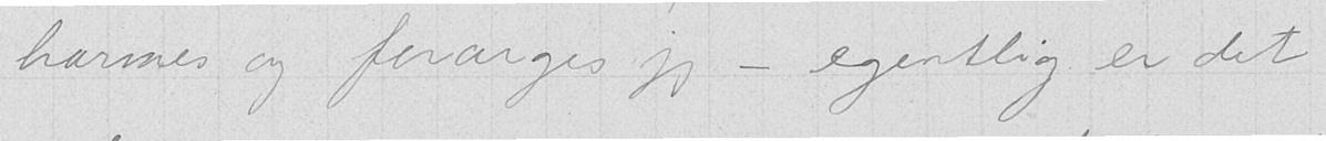harmes og forarges jeg - egentlig er det
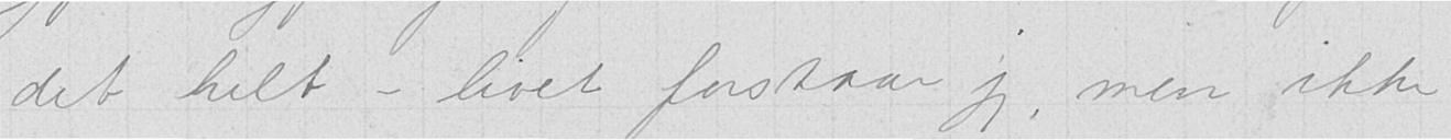det helt - livet forstaar jeg, men ikke
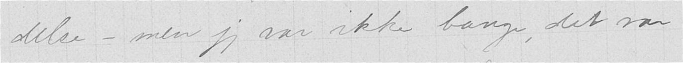delse - men jeg var ikke bange, det var
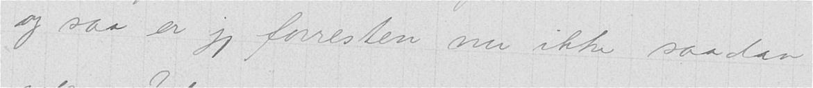og saa er jeg forresten nu ikke saadan
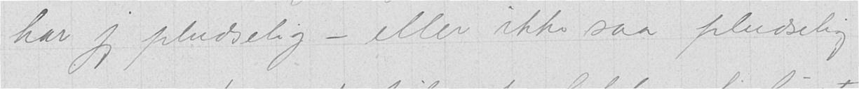har jeg pludselig - eller ikke saa pludselig
